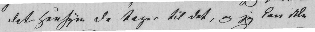det Hensyn De tager til det, og jeg kan ikke
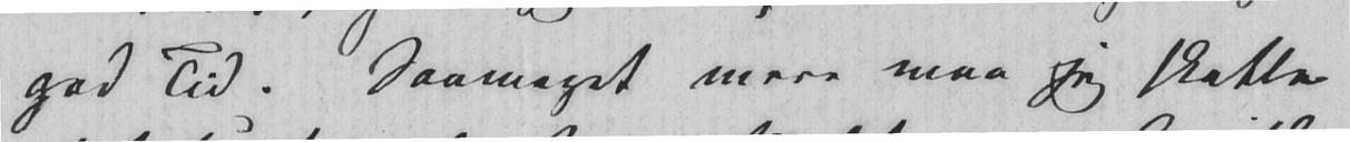god Tid. Saameget mere maa jeg skatte
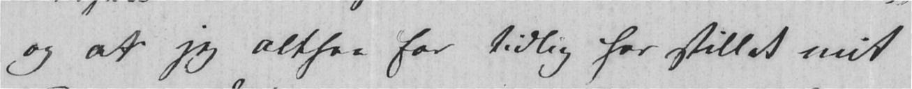og at jeg altsaa for tidlig har stillet mit
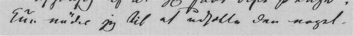kun nødes jeg til at udsætte den noget.
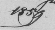1859
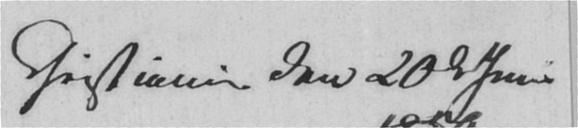Christiania den 20de Juni
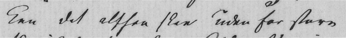Kan det altsaa skee uden for store
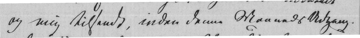og mig tilsendt, inden denne Maaneds Udgang.
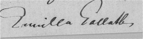Camilla Collett
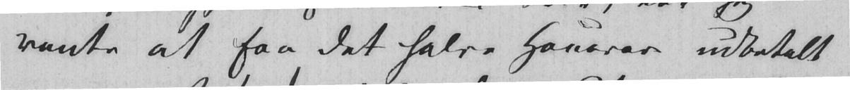vente at faa det halve Honorar udbetalt
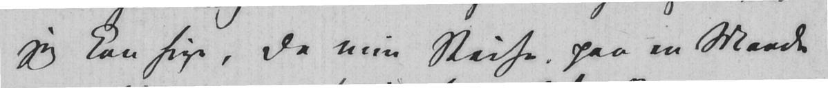jeg kan sige, da min Reise paa en Maade
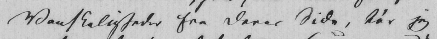Vanskeligheder fra Deres Side, tør jeg
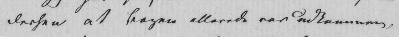derhen at Bogen allerede var udkommen,
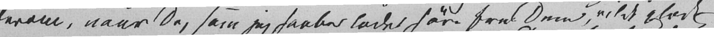derom, naar De, som jeg haaber lader høre fra Dem, vilde glæde
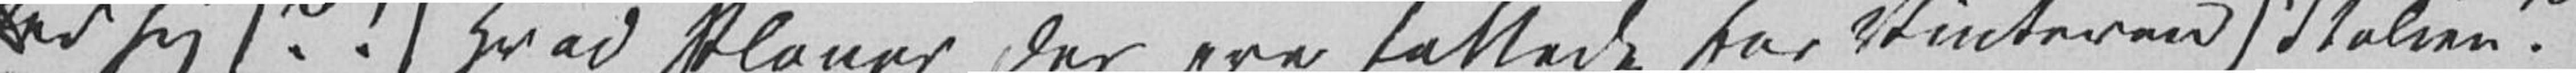der sig (?!) Hvad Planer der ere fattede for Vinteren (Italien?
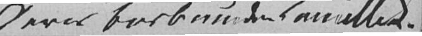Deres forbundne Camilla C.
